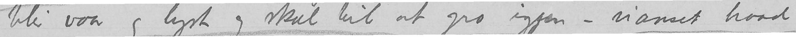blir vaar og lyst og skal til at gro igjen – uanset hvad
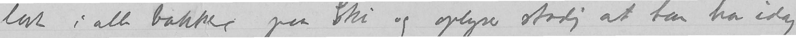lavt i alle bakker paa Ski og oplyser stadig at han har idag
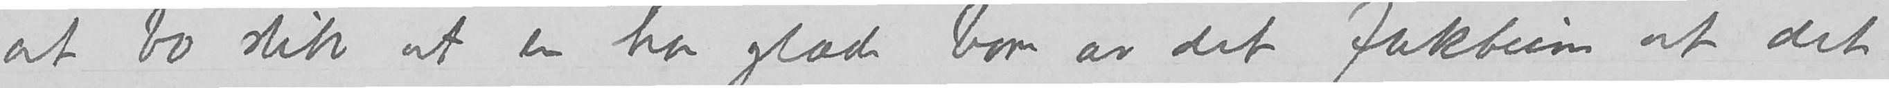at bo slik at en har glæde bare av det faktum at det
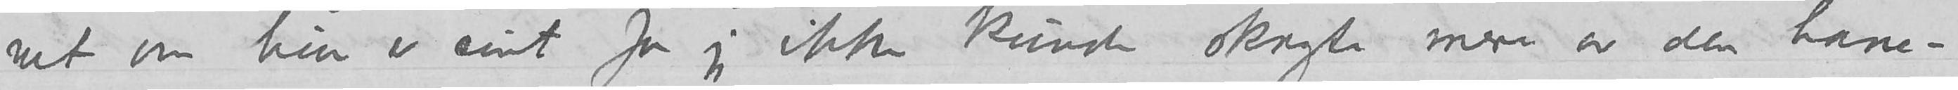vet om hun er sint for jeg ikke kunde skryte mere av den hane-
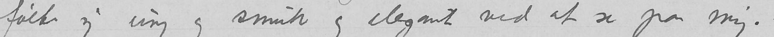følte sig ung og smuk og elegant ved at se paa mig. –
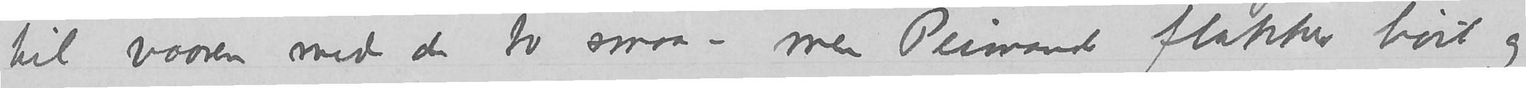til vaaren med de to smaa – men Peimand flakker høit og
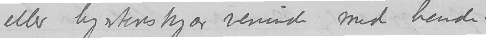eller hjertenskjær veninde med hende.
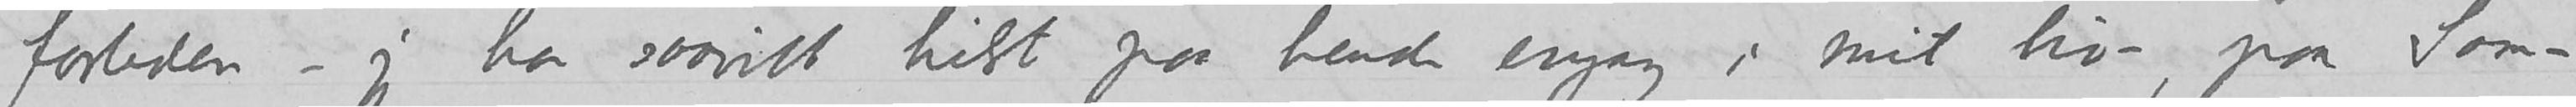forleden – jeg har saavidt hilst paa hende engang i mit liv, paa Sam-
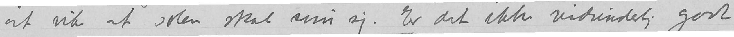at vite at solen skal snu sig. Er det ikke vidunderlig godt
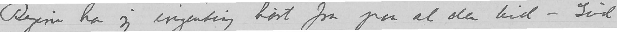Regine har jeg ingenting hørt fra paa al den tid – Gud
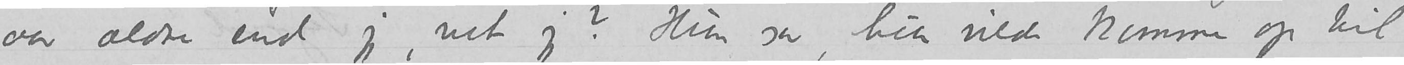aar ældre end jeg, vet jeg? Hun sa, hun vilde komme op til
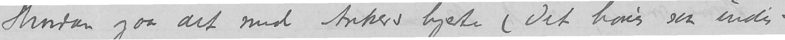Hvordan gaar det med Ankers hjerte (Det høres saa indi-
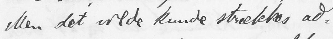Men det vilde kunde strækkes at-
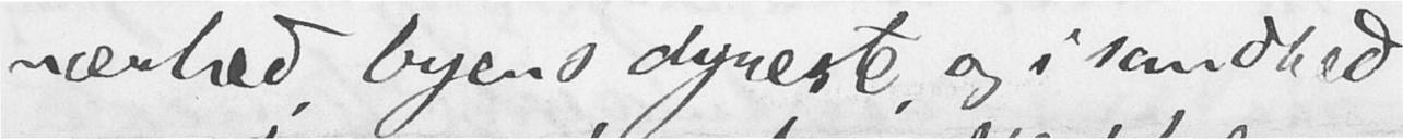nærhed, byens dyreste, og i sandhed
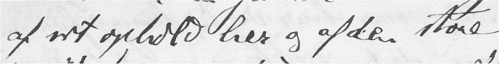af sit ophold her og af den store
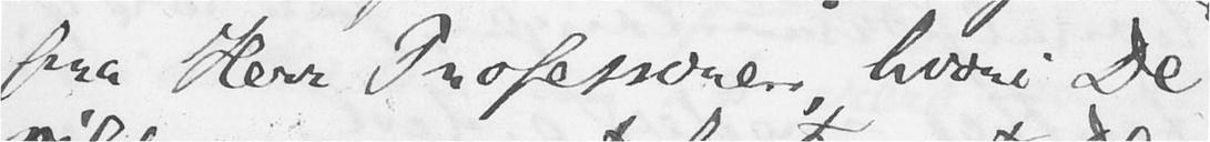fra Herr Professoren, hvori De
